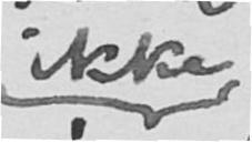ikke
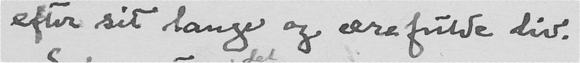efter sit lange og ærefulde liv.
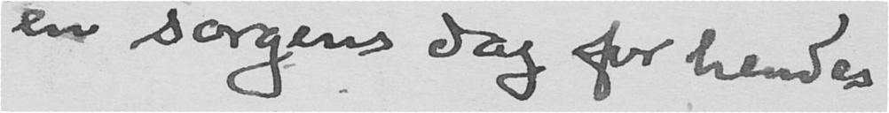en sorgens dag for hendes
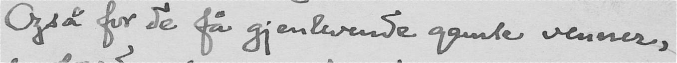Også for de få gjenlevende gamle venner,
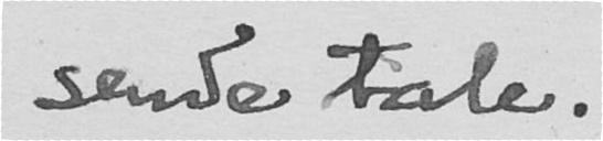sende tale.
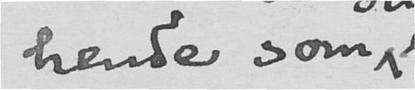hende som
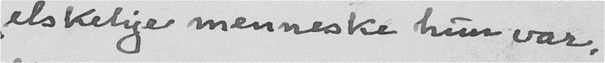elskelige menneske hun var.
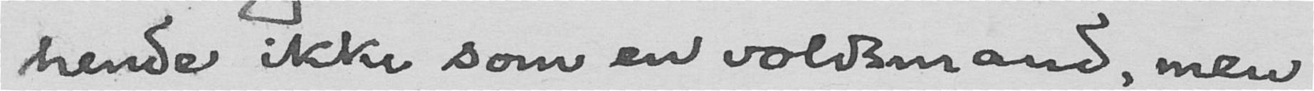hende ikke som en voldsmand, men
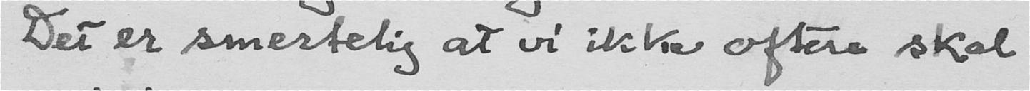Det er smertelig at vi ikke oftere skal
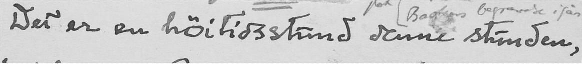Det er en høitidsstund denne stunden,
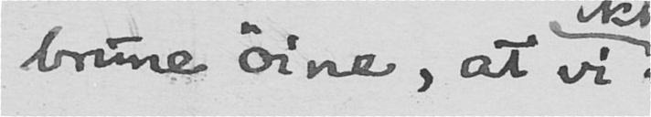brune øine, at vi
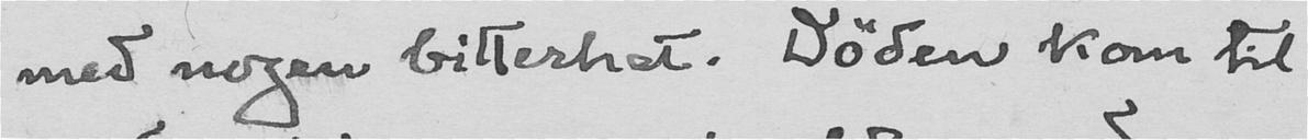med nogen bitterhet. Døden kom til
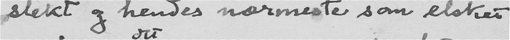slekt og hendes nærmeste som elsket
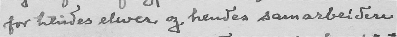for hendes elever og hendes samarbeidere
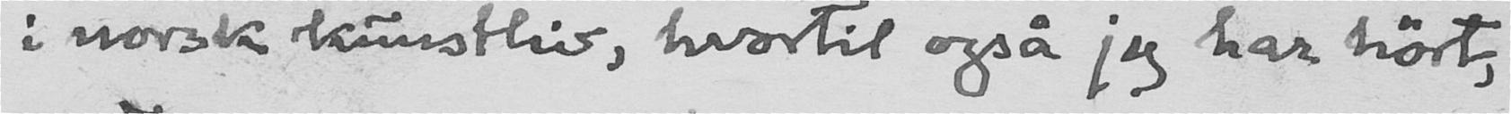i norsk kunstliv, hvortil også jeg har hørt,
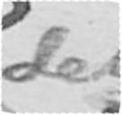det
